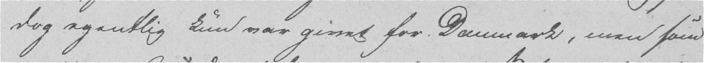dog egentlig kun var givet for Danmark, men som
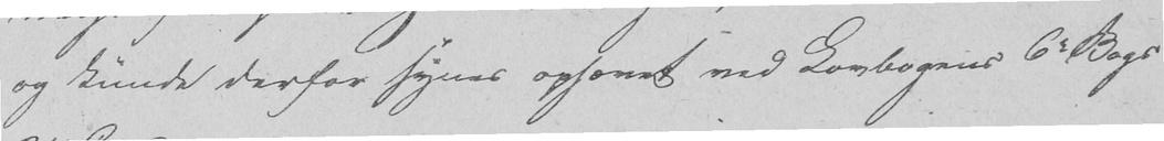og kunde derfor synes ophævet ved Lovbogens 6e Bogs
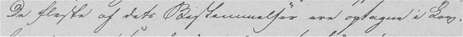De fleste af dets Bestemmelser ere optagne i Lov-
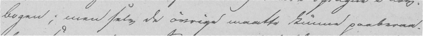bogen; men selv de øvrige maatte kunne paaberaa-
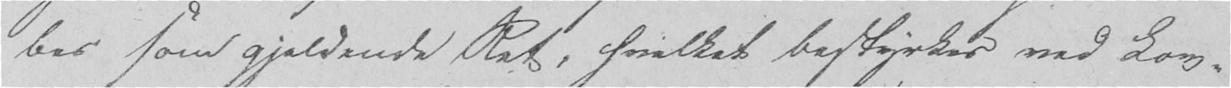bes som gjeldende Ret, hvilket bestyrkes ved Lov-
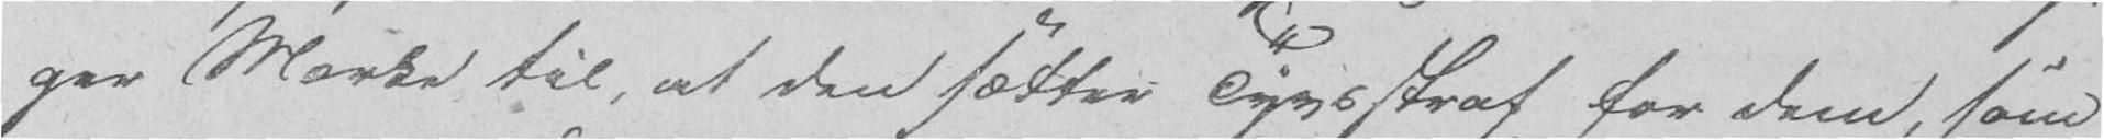ger Mærke til, at den sætter Tyvsstraf for dem, som
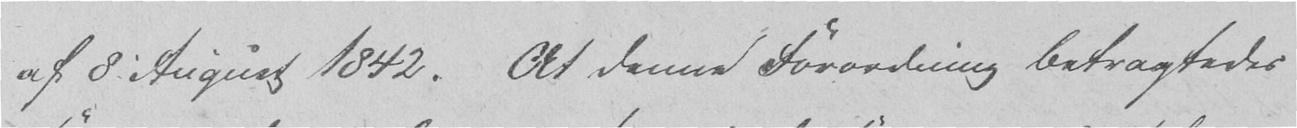af 8 August 1842. At denne Forordning betragtedes
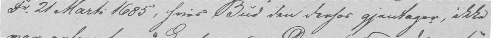Fr. 21 Marti 1685, hvis Bud den derhos gjentager, ikke
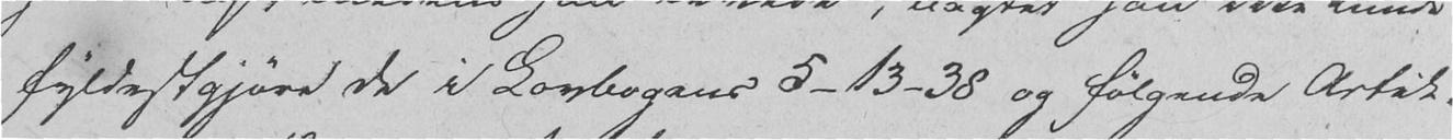fyldestgjøre de i Lovbogens 5-13-38 og følgende Artik-
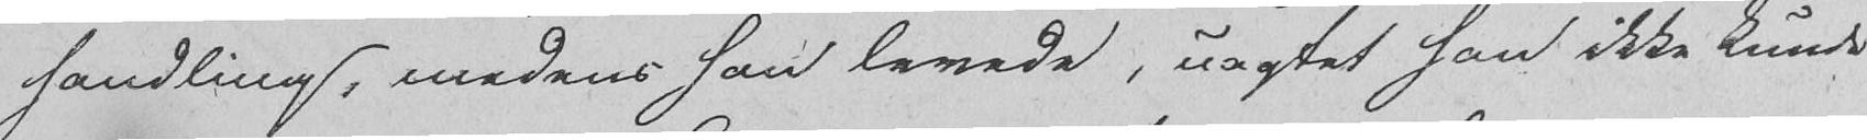handling, medens han levede, uagtet han ikke kunde
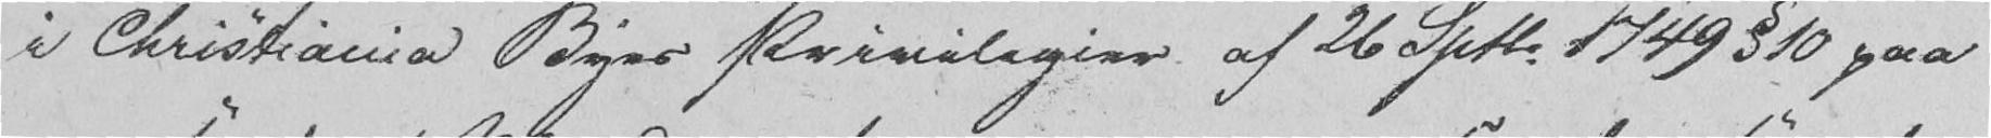i Christiania Byes Privilegier af 26 Sptbr. 1749 § 10 paa
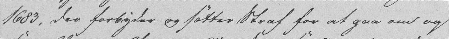1683, der forbyder og sætter Straf for at gaa om og
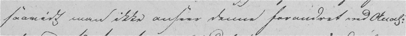saavidt man ikke anseer denne forandret ved Anord.
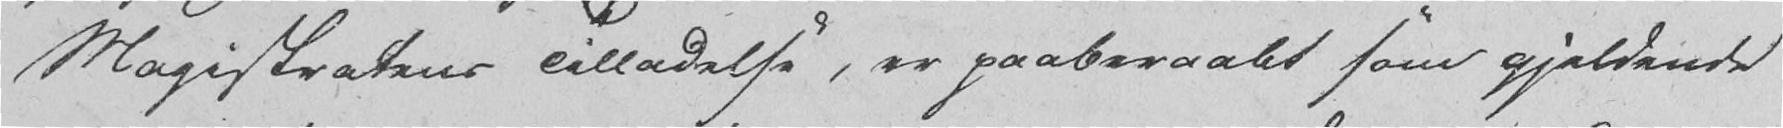Magistratens Tilladelse, er paaberaabt som gjeldende
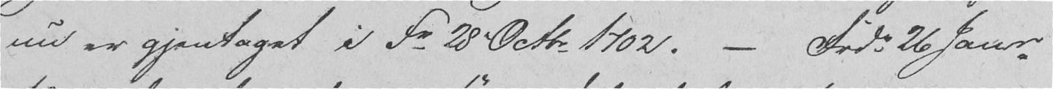nu er gjentaget i Fr 28 Octbr 1702. - Frdn 26 Janr
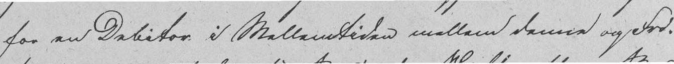for en Debitor i Mellemtiden mellem denne og Frd.
